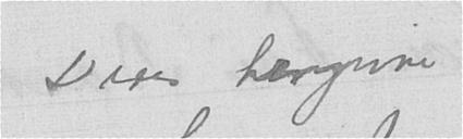Deres hengivne
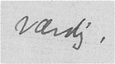værdig.
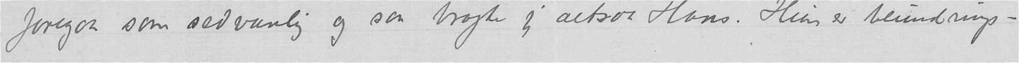foregaa som sedvanlig og saa bragte jeg altsaa Hans. Hun er beundrings-
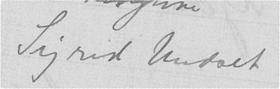Sigrid Undset
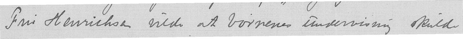Fru Henrichsen vilde at børnenes undervisnig skulde
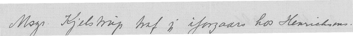Msgr. Kjelstrup traf jeg iforgaars hos Henrichsens.
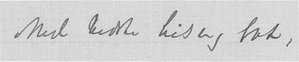Med bedste hilsen og tak,
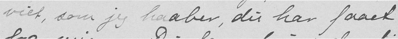viet, som jeg haaber du har faaet
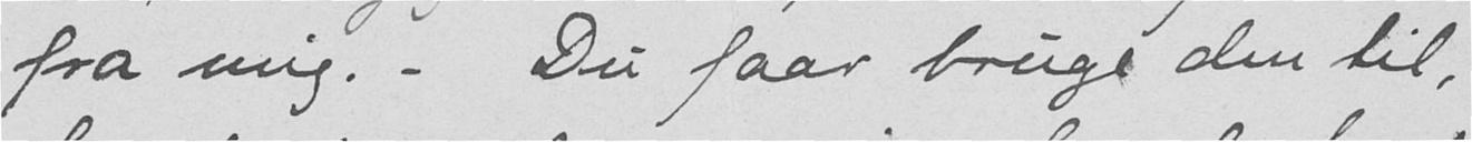fra mig.- Du faar bruge den til
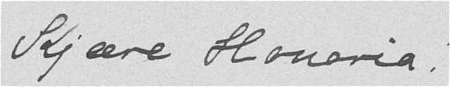Kjære Honoria.
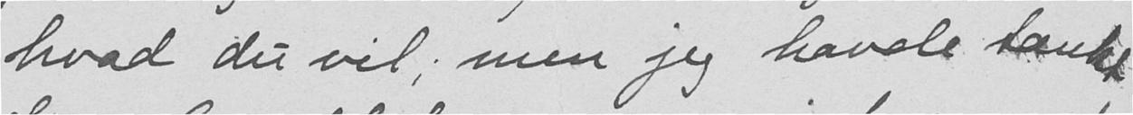hvad du vil men jeg havde tænkt
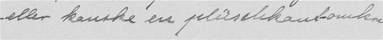eller kanske en pluselekant omkring
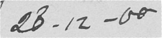28-12-00
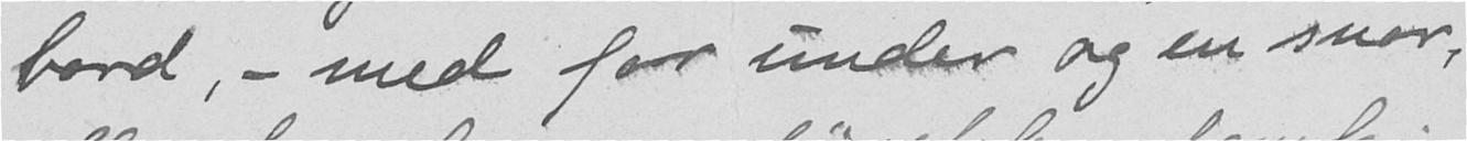bord - med for under og en snor,
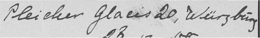Pleicher Glacis 20, Würzburg
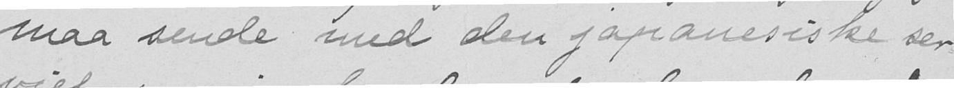maa sende med den japanesiske ser
